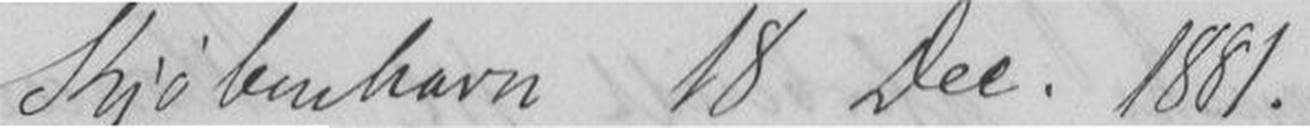Kjøbenhavn 18 Dec. 1881
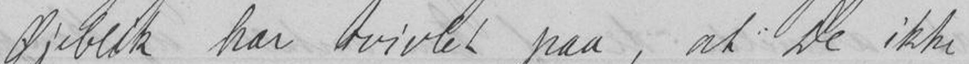Øjeblik har tvivlet paa, at De ikke
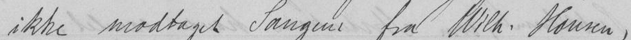ikke modtaget Sangene fra Wilh. Hansen,
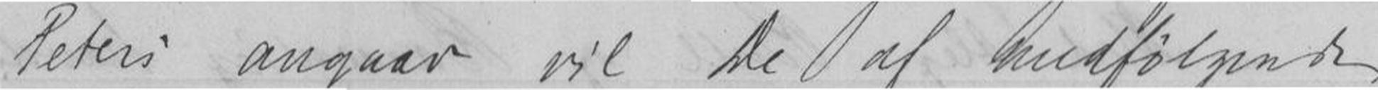Peters angaar vil De af medfølgende
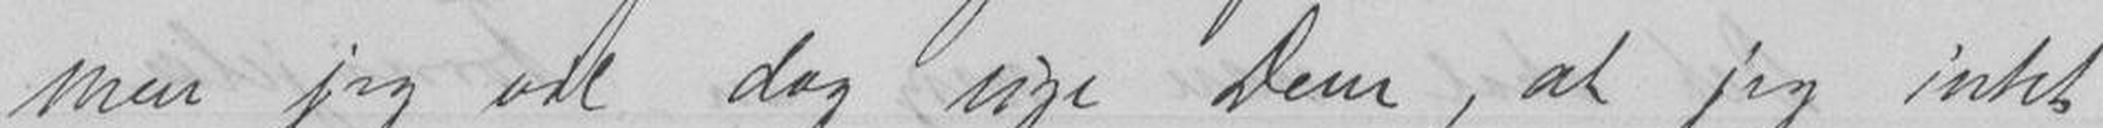men jeg vil dog sige Dem, at jeg intet
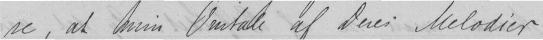se, at min Omtale af Deres Melodier
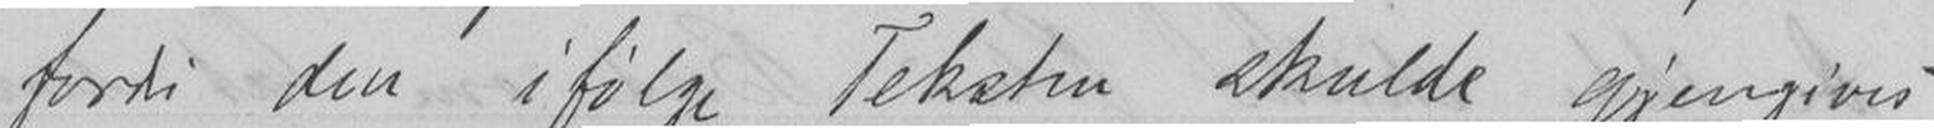fordi den ifølge Teksten skulde gjengives
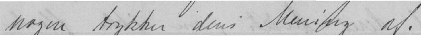nogen trykker dens Mening af.
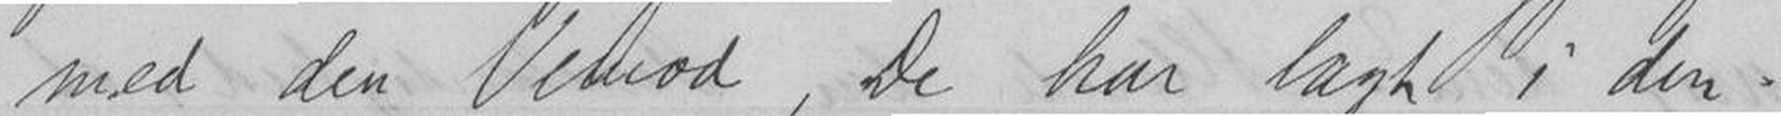med den Vemod, De har lagt i den.
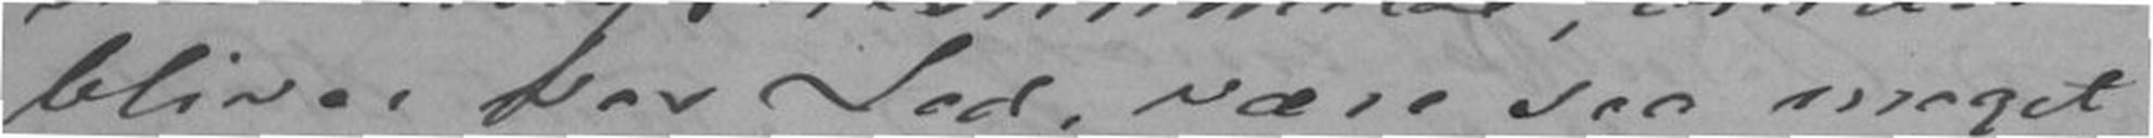bliver vor Lod, være saa meget
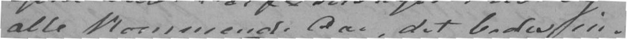alle kommende Aar, det bedes in-
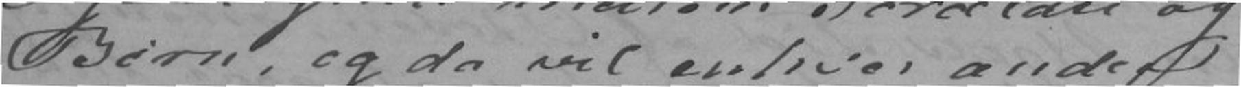Børn, og da vil enhver anden
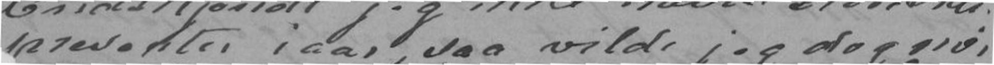presenter i aar, saa vilde jeg dog nø-
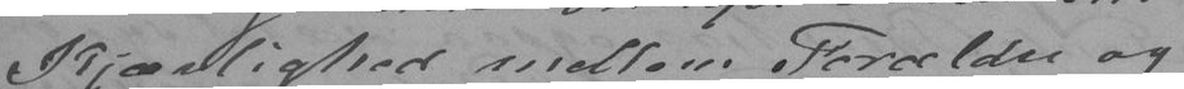Kjærlighed mellem Forældre og
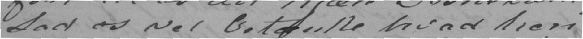Lad os vel betænke hvad heri
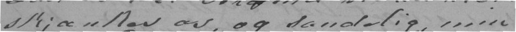skjænkes os, og sandelig, min
